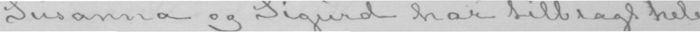Susanna og Sigurd har tillbragt hele
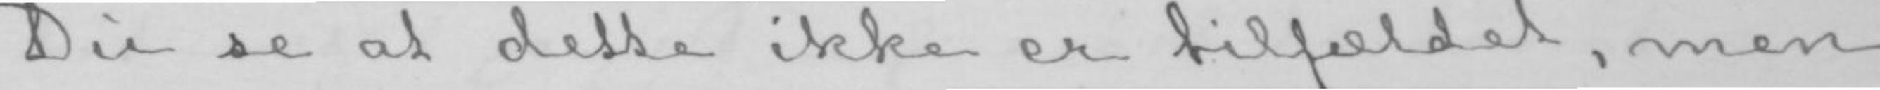Du se at dette ikke er tilfældet, men
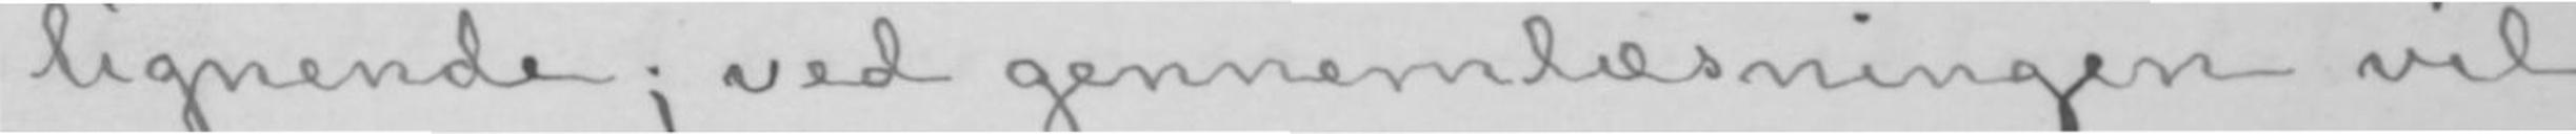lignende; ved gennemlæsningen vil
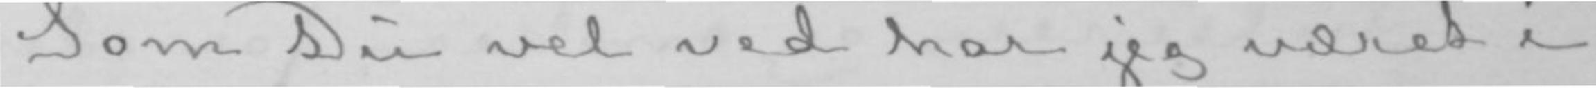Som Du vel ved har jeg været i
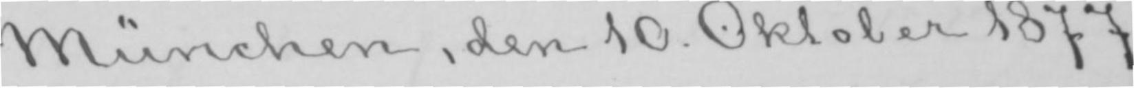München, den 10. Oktober 1877.
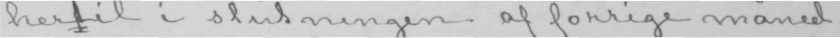herftil i slutningen af forrige måned.
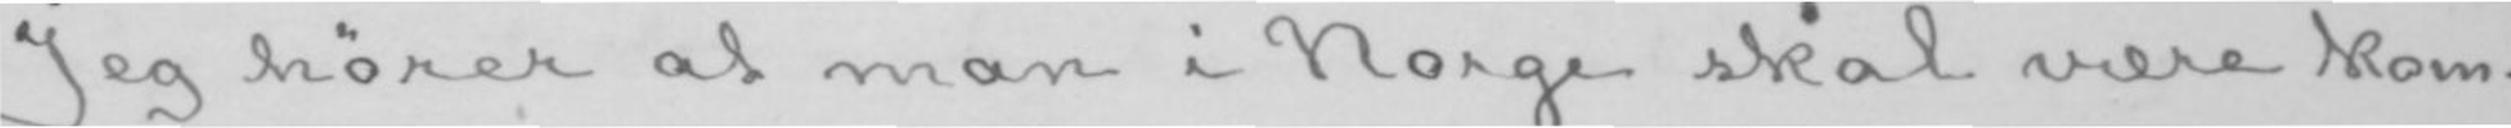Jeg hører at man i Norge skal være kom-
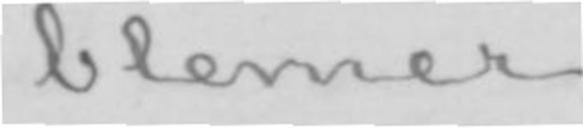blemer.
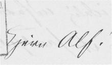Kjere Alf!
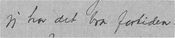jeg har det bra fortiden.
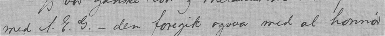med A.E.G. — den foregik ogsaa med al honnør
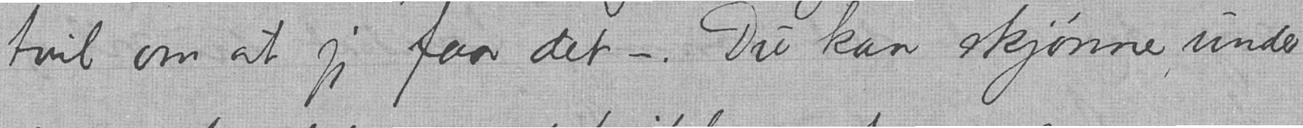tvil om at jeg faar det —. Du kan skjønne, under
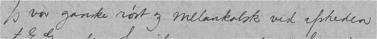Jeg var ganske rørt og melankolsk ved afskeden
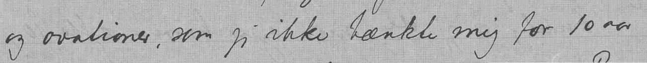og ovationer, som jeg ikke tænkte mig for 10 aar
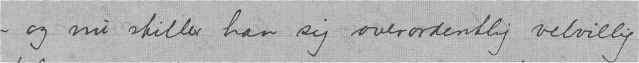— og nu stiller han sig overordentlig velvillig
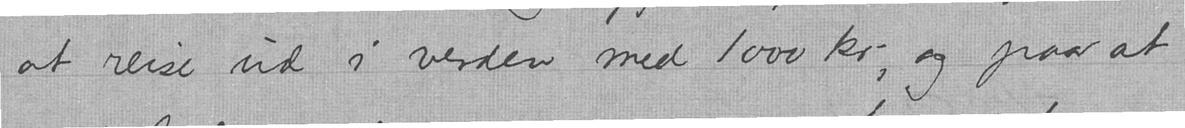at reise ud i verden med 1000 kr; og paa at
